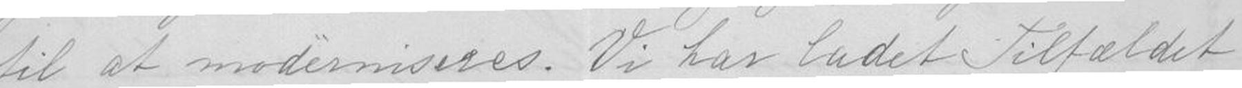til at moderniseres. Vi har ladet Tilfældet
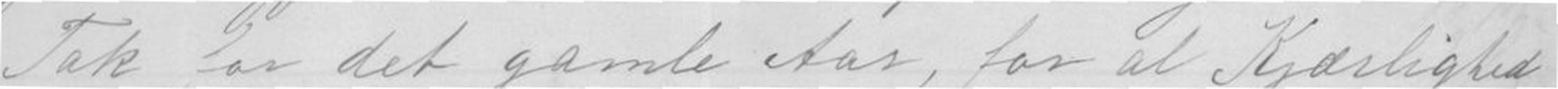Tak for det gamle Aar, for al Kjærlighed
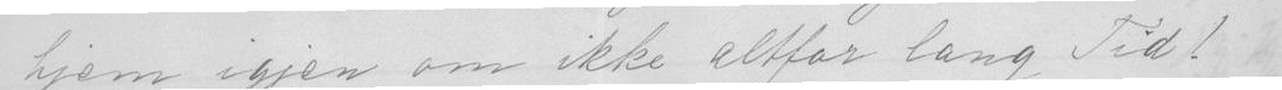hjem igjen om ikke altfor lang Tid!
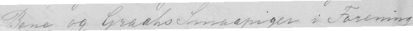Bene og Graahs Smaapiger i Forening.
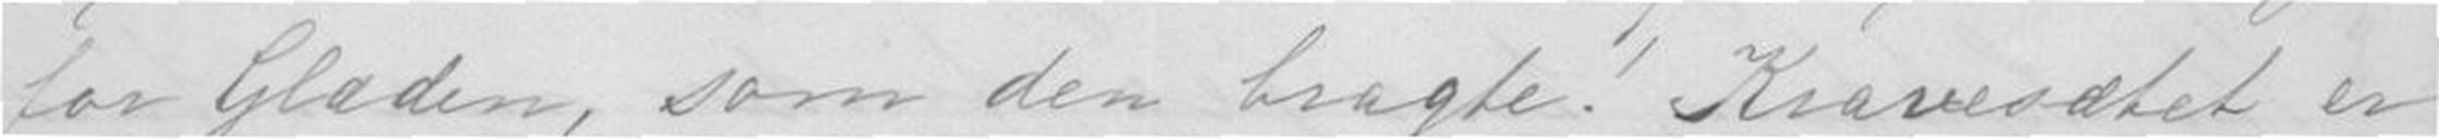for Glæden, som den bragte! Kravesætet er
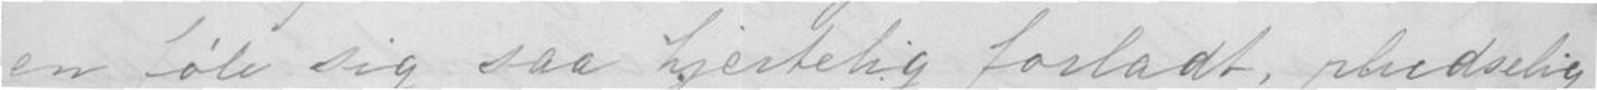en føle sig saa hjertelig forladt, pludselig
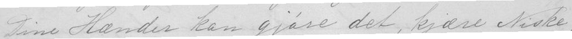Dine Hænder kan gjøre det, Kjære Niske,
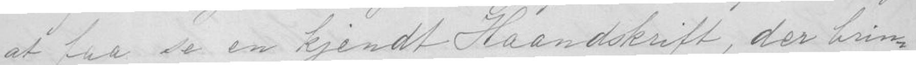at faa se en kjendt Haandskrift, der brin-
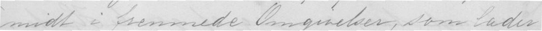midt i fremmede Omgivelser, som lader
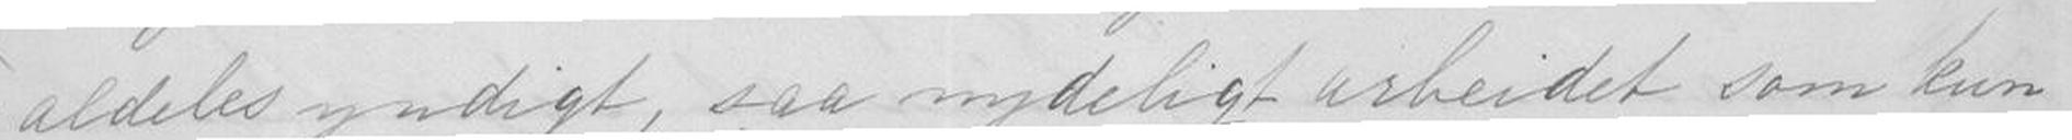aldeles yndigt, saa nydeligt arbeidet som kun
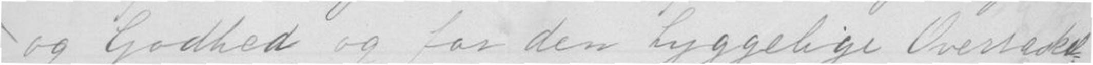og Godhed og for den hyggelige overraskel-
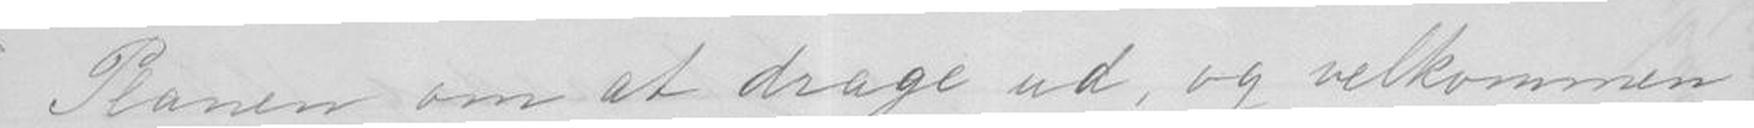Planen om at drage ud, og velkommen
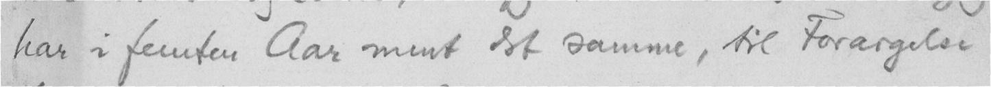har i femten Aar ment det samme, til Forargelse
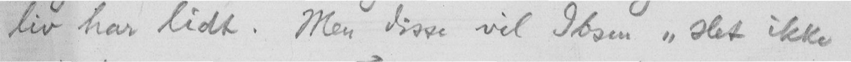liv har lidt. Men disse vil Ibsen "slet ikke
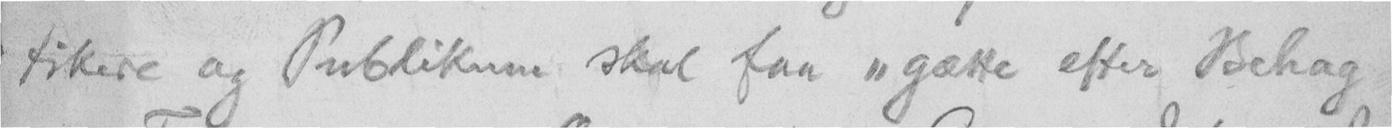tikere og Publikum skal faa "gætte efter Behag
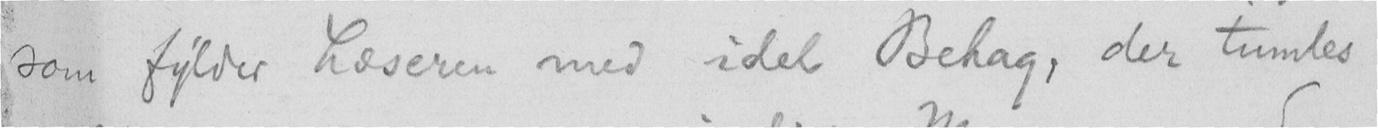som fylder Læseren med idel Behag, der tumles
